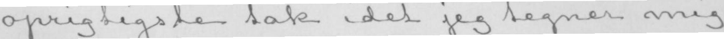oprigtigste tak idet jeg tegner mig
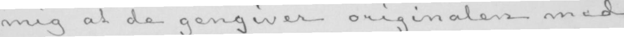mig at de gengiver originalen med
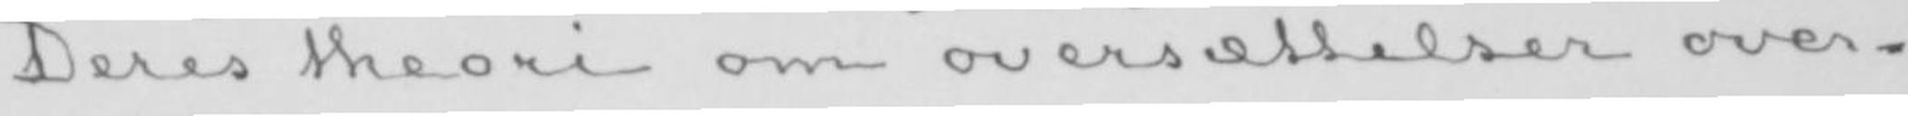Deres theori om oversættelser over-
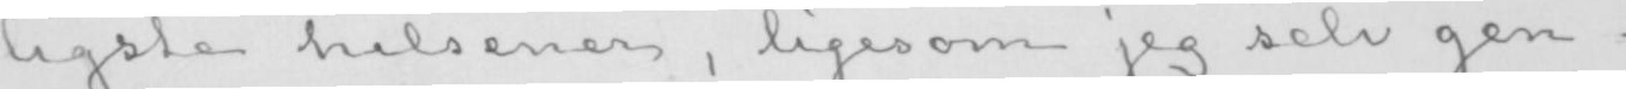ligste hilsener, ligesom jeg selv gen-
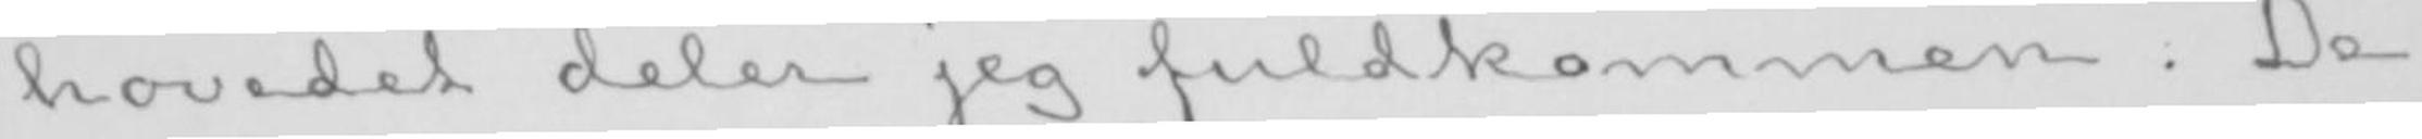hovedet deler jeg fuldkommen. De
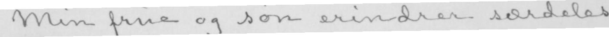Min frue og søn erindrer særdeles
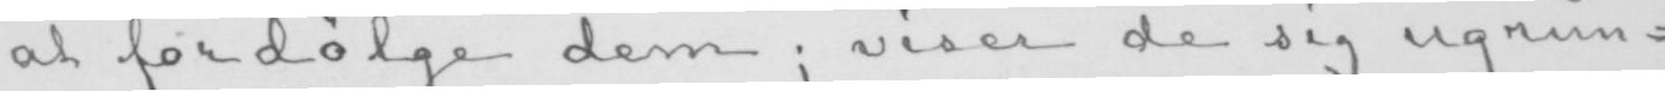at fordølge dem; viser de sig ugrun-
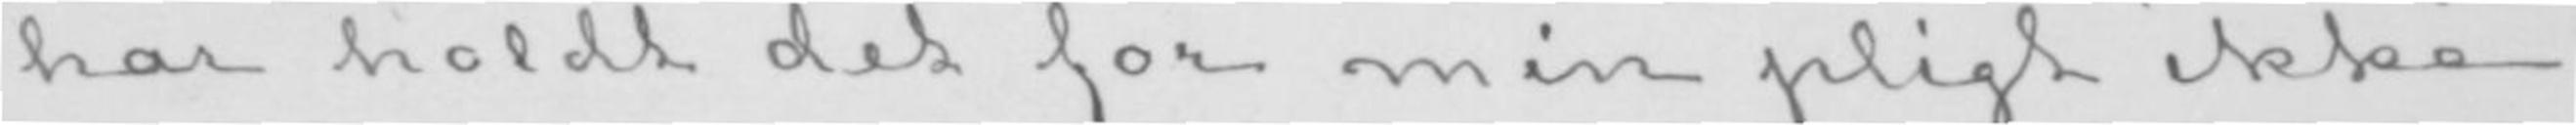har holdt det for min pligt ikke
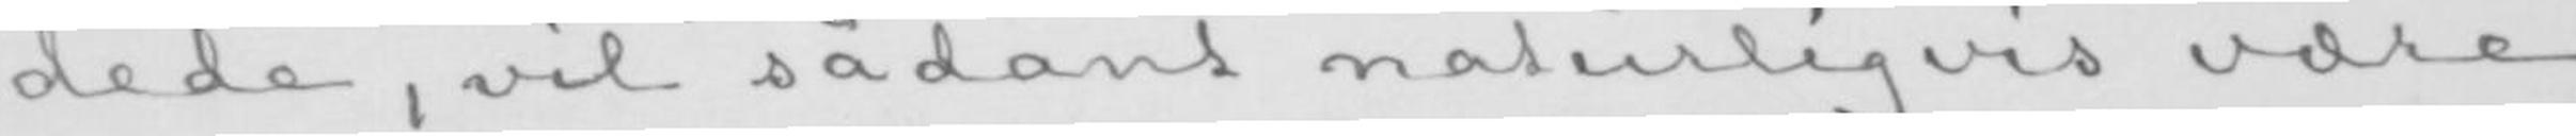dede, vil sådant naturligvis være
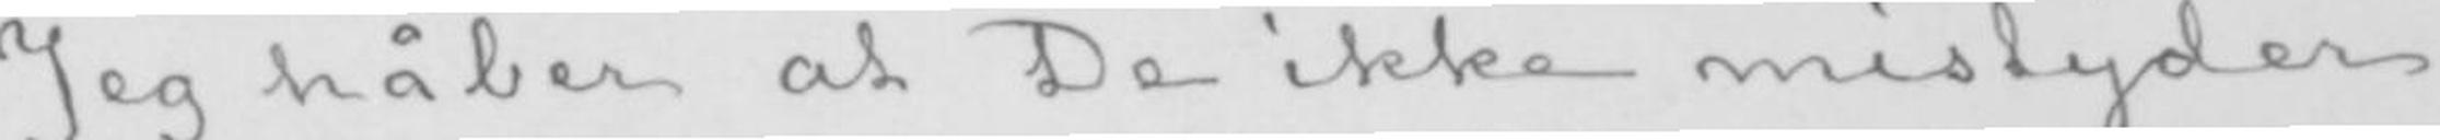Jeg håber at De ikke mistyder
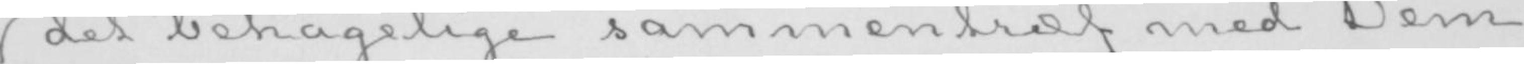det behagelige sammentræf med Dem
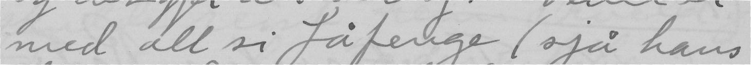med all si fåfenge (sjå hans
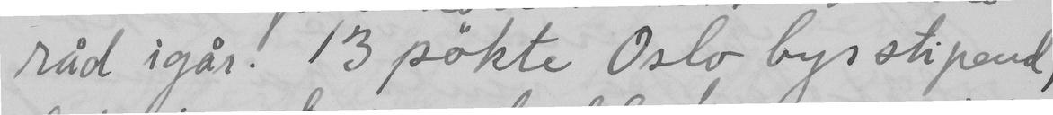råd igår. 13 sökte Oslo bys stipend,
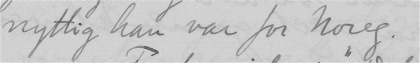nyttig han var for Noreg.
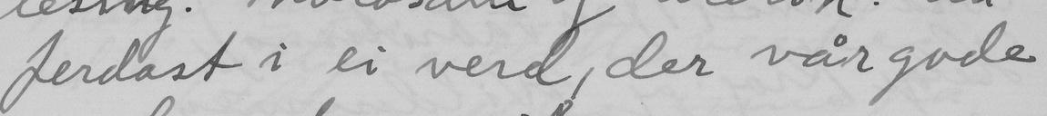ferdast i ei verd, der vår gode
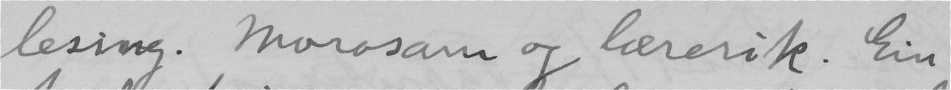lesing. Morosam og lærerik. Ein
